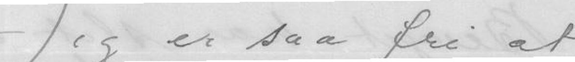Jeg er saa fri at
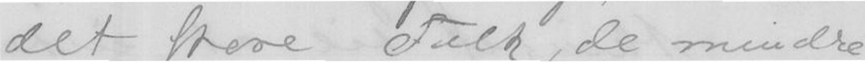det store Folk, de mindre
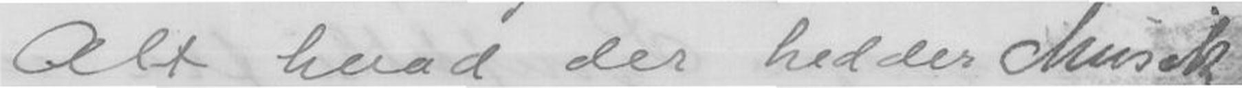Alt hvad der hedder Musik
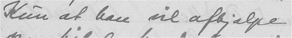Kun at han vil afhjælpe
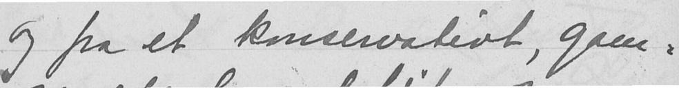Og fra et konservativt, gam-
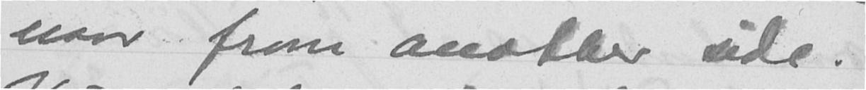naar from another side.
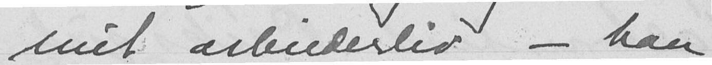mit arbeiderliv - han
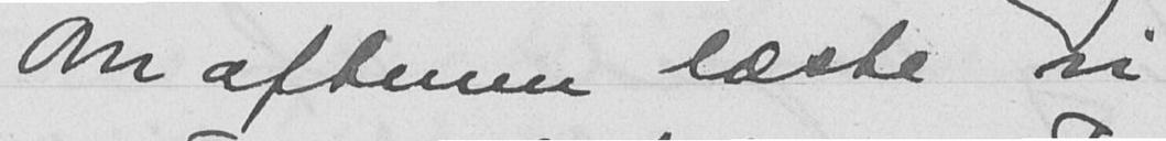Om aftenen læste vi
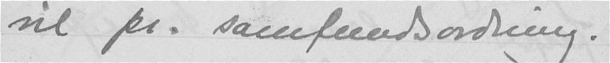vil pr. samfundsordning.
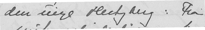den unge Hertzberg: Fra
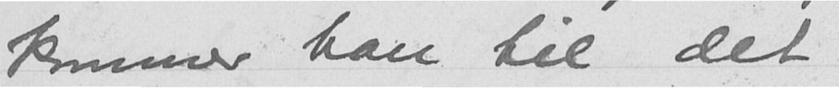kommer han til det
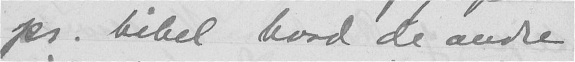pr. bibel hvad de andre
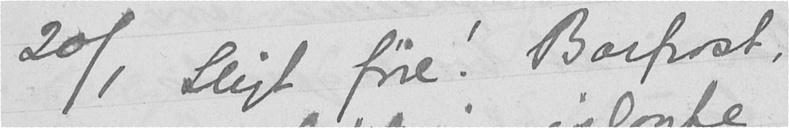20/1 Sligt føre! Barfrost,
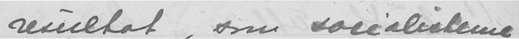resultat, som socialisterne
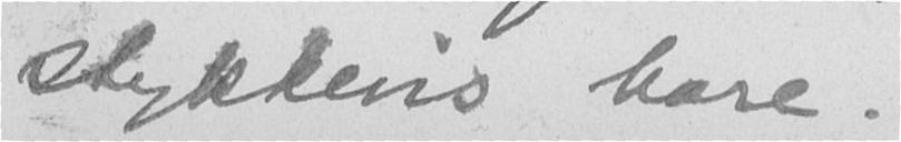stykkevis bare.
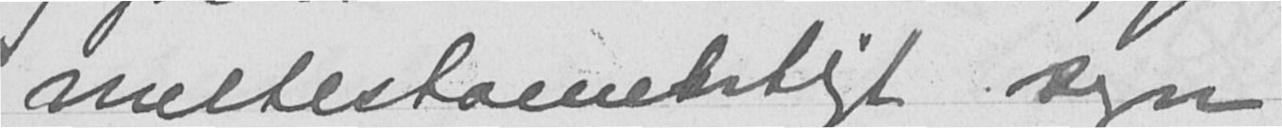meltestamentligt syn
